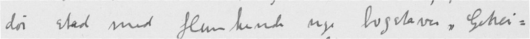dør stod med flunkende nye bogstaver "Gehei-
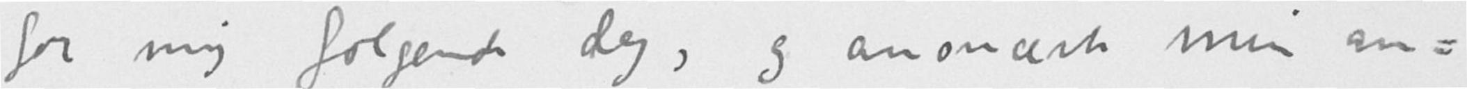for mig følgende dag, og anoncerte min an-
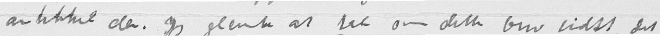artikkel der. Jeg glemte at tale om dette brev sidst, det
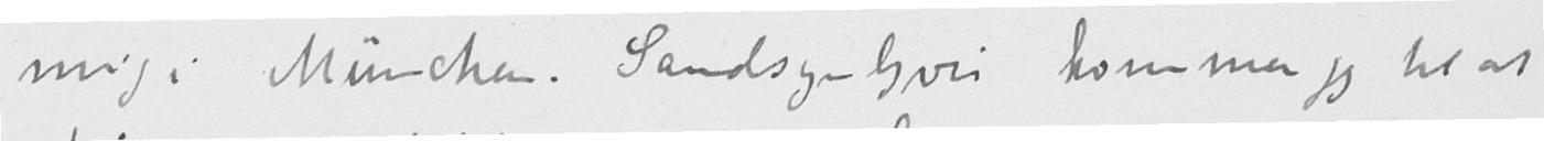mig i München. Sandsynligvis kommer jeg til at
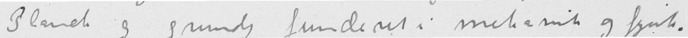Planck og grundig funderet i mekanik og fysik.
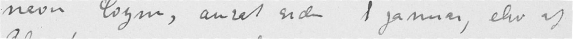navn Coym, ansat siden 1 januar, elev af
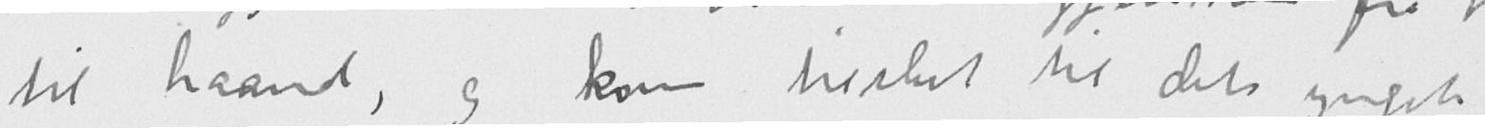til haand, og kom tilslut til dets yngste
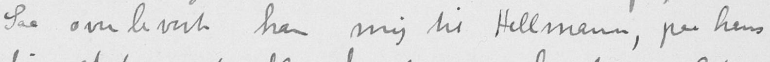Saa overleverte han mig til Hellmann, paa hans
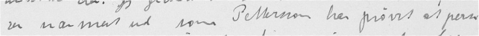ser nærmest ud som Pettersson har prøvet at presse
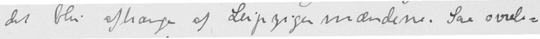det blir afhænger af Leipzigermændene. Saa overle-
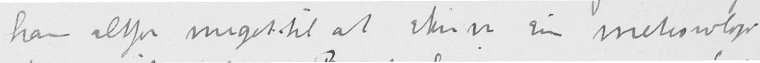ham altfor meget til at skrive sin meteorologi
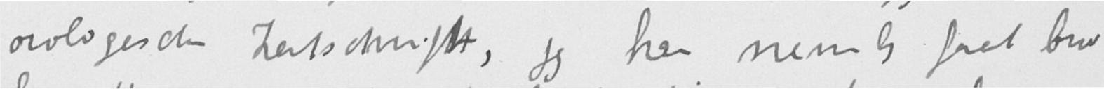orologische Zeitschrift, jeg har nemlig faat brev
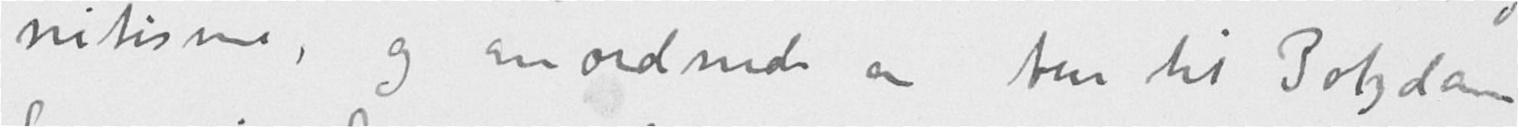nitisme, og anordnede en tur til Potzdam
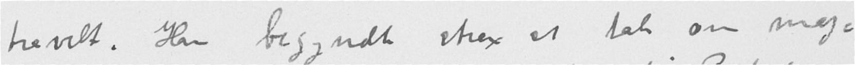travelt. Han begyndte strax at tale om mag-
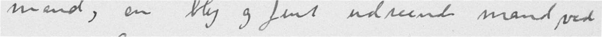mand, en bleg og fint udseende mand ved
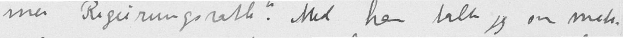mer Regierungsrat." Med ham talte jeg om mete-
